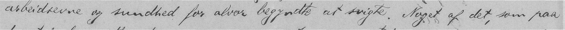arbeidsevne og sundhed for alvor begyndte at svigte. Noget af det, som paa
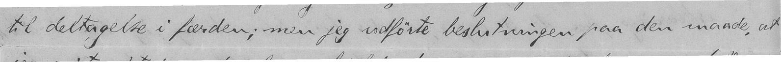til deltagelse i færden; men jeg udførte beslutningen paa den maade, at
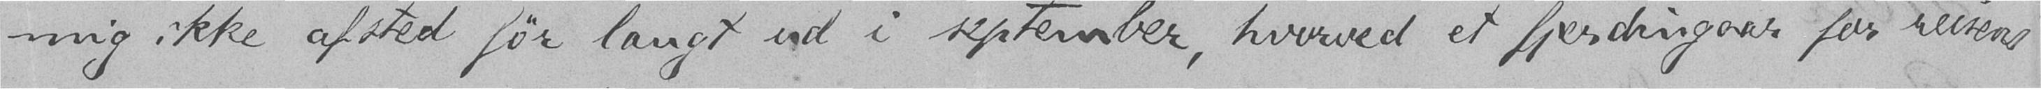mig ikke afsted før langt ud i september, hvorved et fjerdingaar for reisens
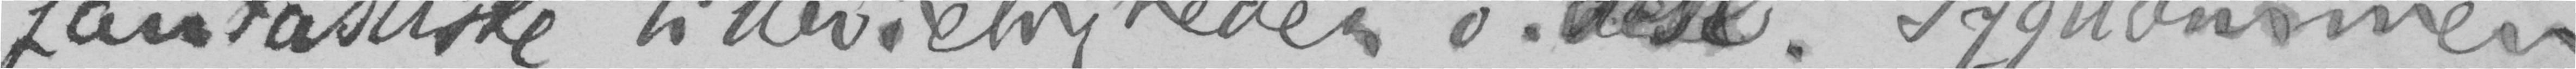fantastiske tilbøieligheder o. desl. Sygdommen,
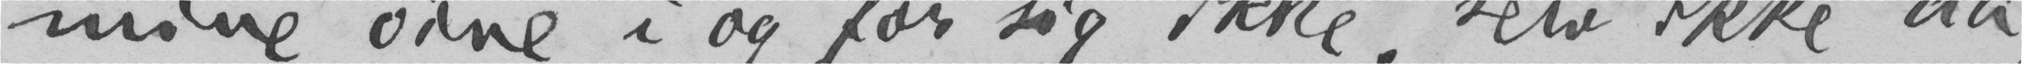mine øine i og for sig ikke, selv ikke da,
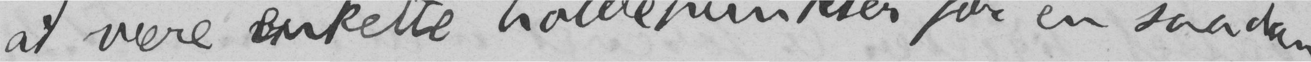at være enkelte holdepunkter for en saadan
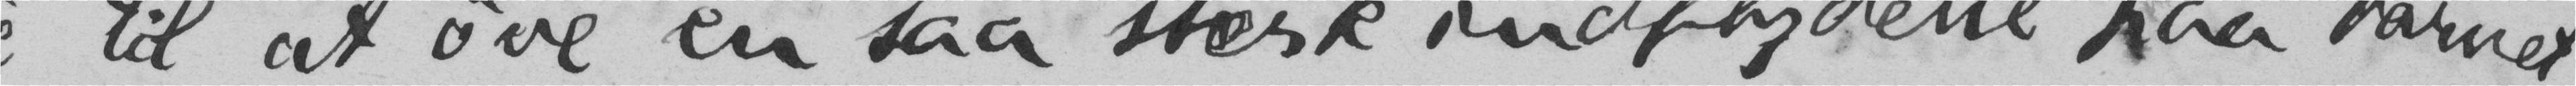til at øve en saa stærk indflydelse paa barnet,
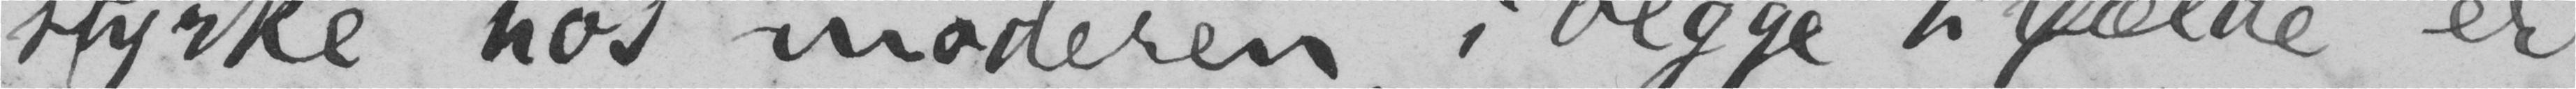styrke hos moderen, i begge tilfælde er
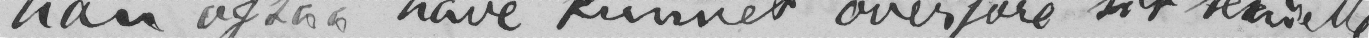han ogsaa have kunnet overføre sit sexuelle
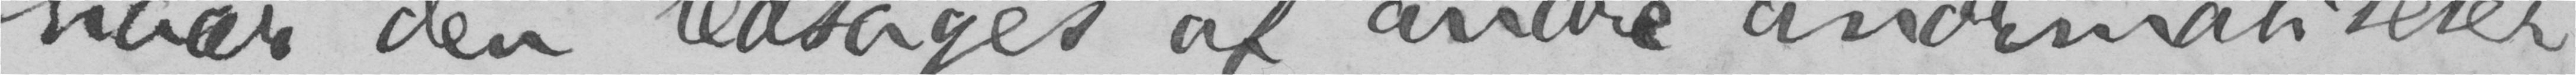naar den ledsages af andre anormaliteter,
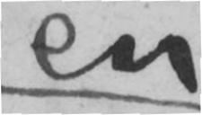en
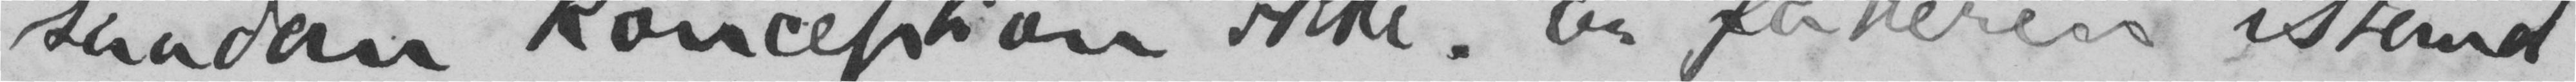saadan konception ikke. Er faderen istand
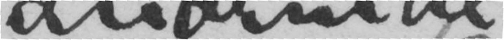anormal
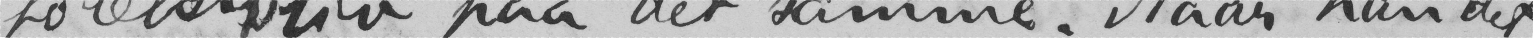følelsesliv paa det samme. Naar han det
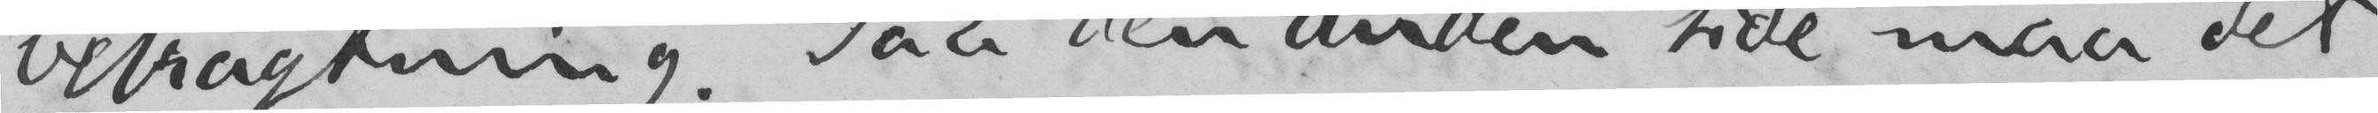betragtning. Paa den anden side maa det
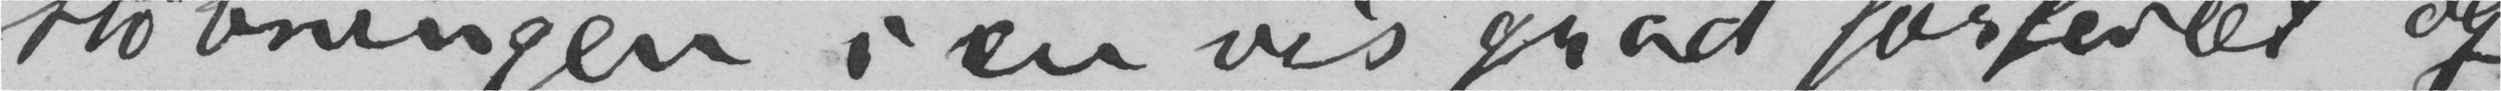støbningen i en vis grad forfeilet og
